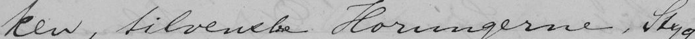ken, tilvenstre Horungerne, Styg-
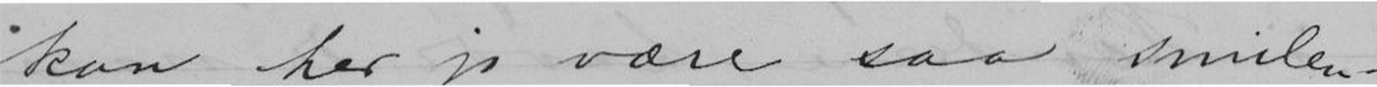kan her jo være saa smilen-
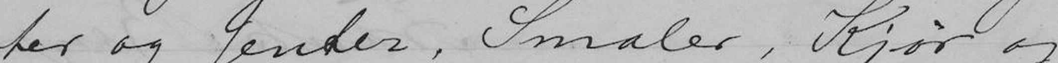ter og Jenter, Smaler, Kjør og
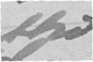thd
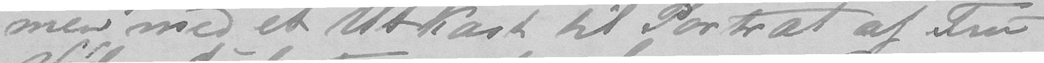men med et Utkast til Portræt af Fru
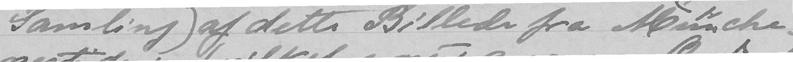Samling) af dette Billede fra Münche-
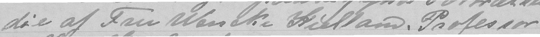die af Fru Wencke Kielland. Professor
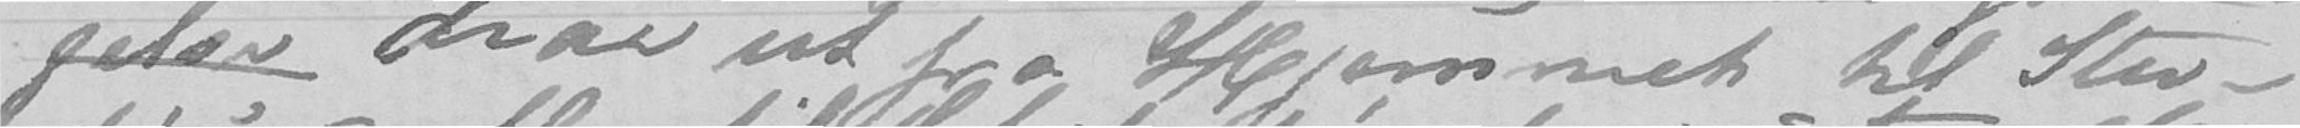gelse drar ut fra Hjemmet til Stu-
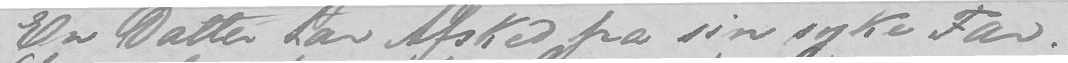En Datter tar Afsked fra sin syke Far.
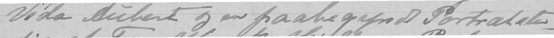Vida Aubert og en paabegyndt Portrætstu-
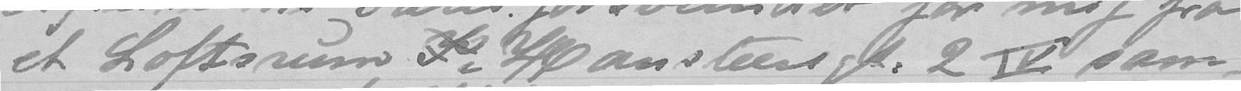et Loftsrum H i Hansteensgt. 2 IV sam-
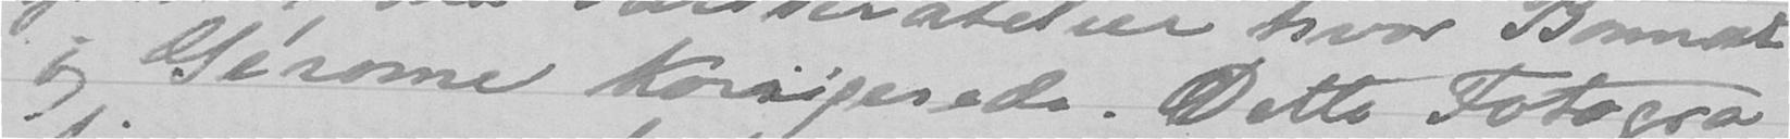og Gérome korrigerede. Dette Fotogra-
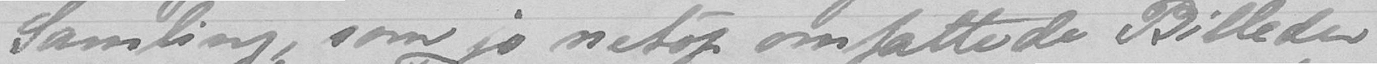Samling, som jo netop omfattede Billeder
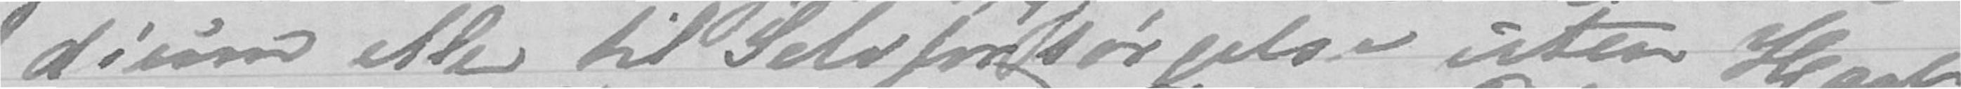dium eller til Selvforsørgelse uten Hast
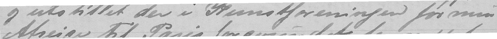og utstillet der i Kunstforeningen før min
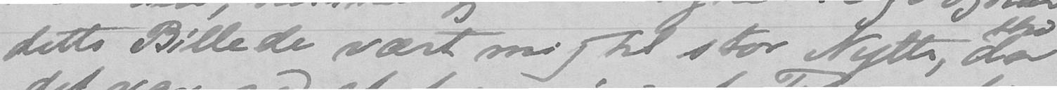dette Billede vært mig til stor Nytte, da
